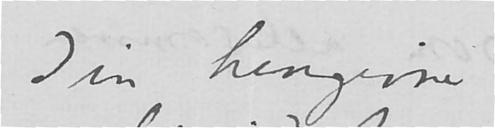Mange hilsener fra Svarstad og mig til ing Anker og dig selv
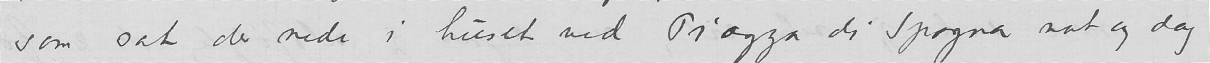som sat der nede i huset ved Piazza di Spagna nat og dag
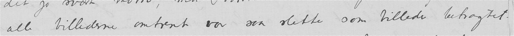alle billederne omtrent var saa slette som billeder betragtet.
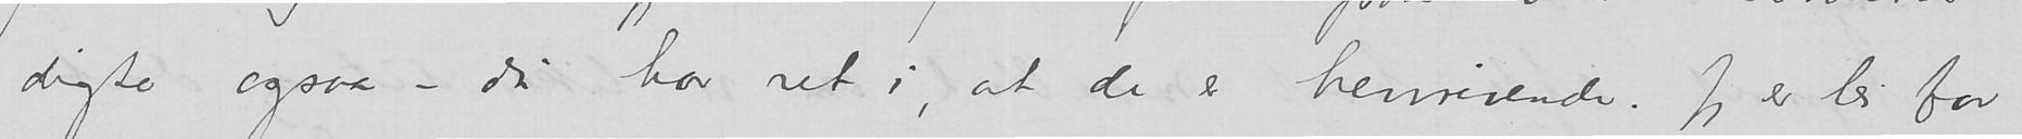digte ogsaa – du har ret i, at de er henrivende. Jeg er lei for
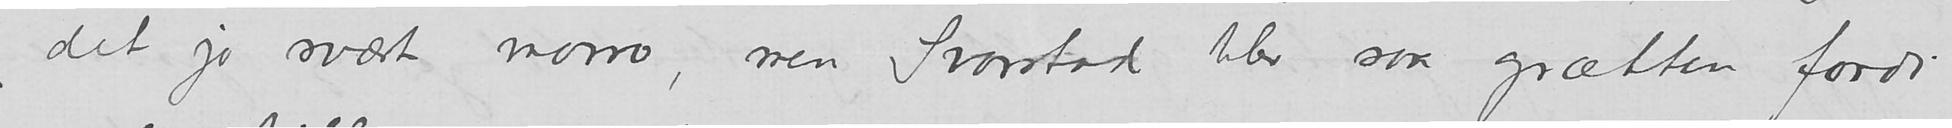det jo svært morro, men Svarstad blev så grætten fordi
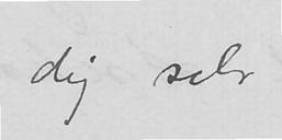dig selv
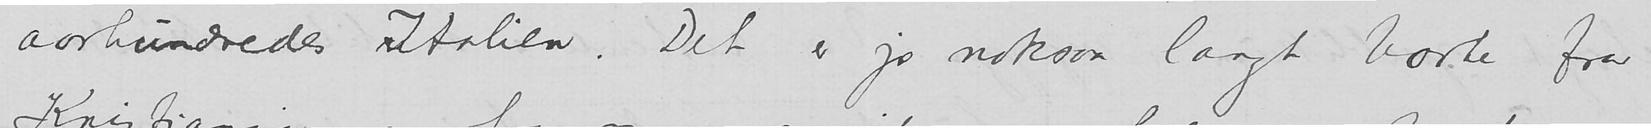aarhundredes Italien. Det er jo noksaa langt borte fra
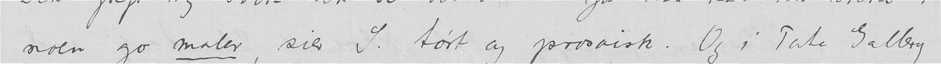noen go maler, sier S. tørt og prosaisk. Og i Tate Gallery
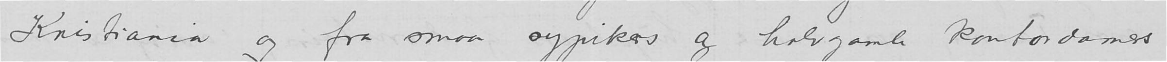Kristiania og fra smaa sypikers og halvgamle kontordamers
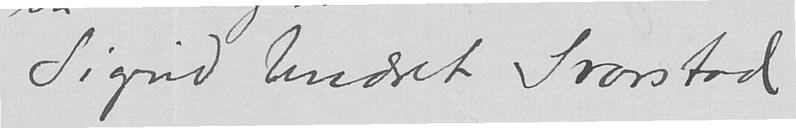Din hengivne
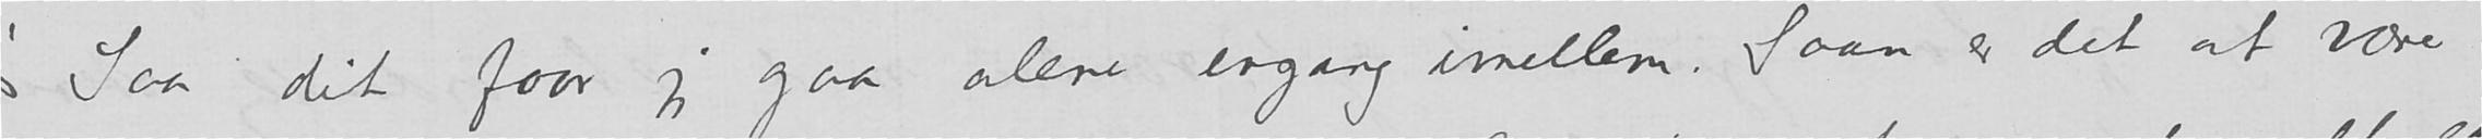Saa dit faar jeg gaa alene engang imellem. Saan er det at være
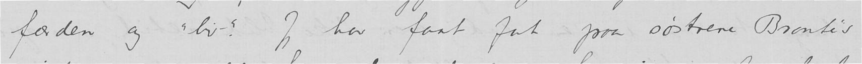færden og «liv». Jeg har faat fat paa søstrene Brontës
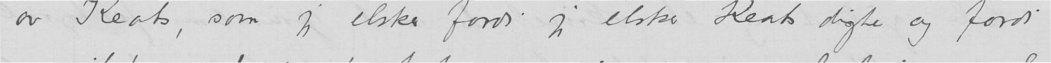av Keats, som jeg elsker fordi jeg elsker Keats digte og fordi
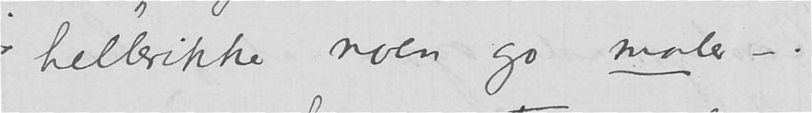hellerikke noen go maler –.
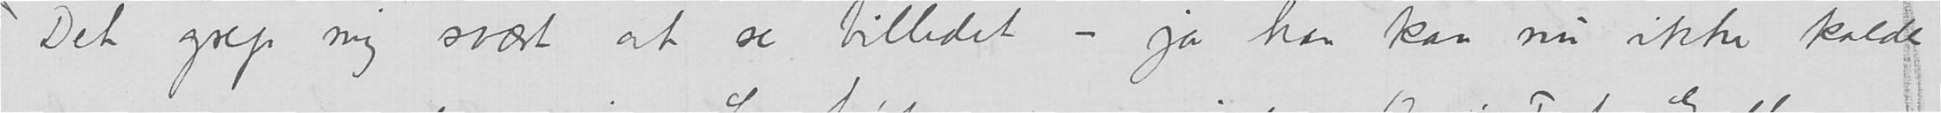Det grep mig svært at se billedet – ja han kan nu ikke kalde
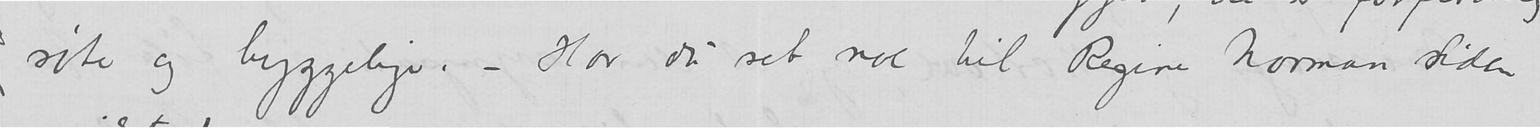søte og hyggelige. – Har du set noe til Regine Norman siden
